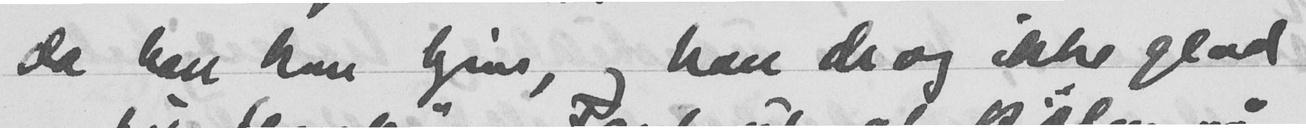da han kom hjem, og han drog ikke glad
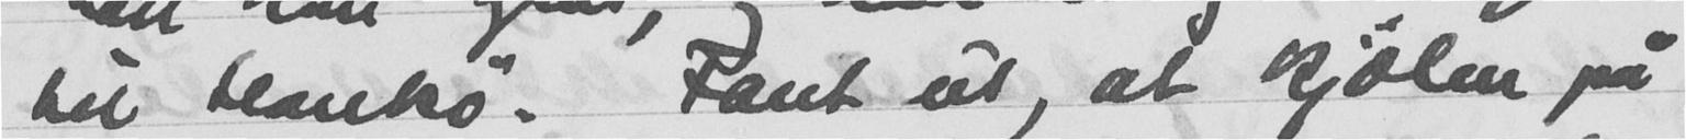til Hankø. Fant ut, at kjølen på
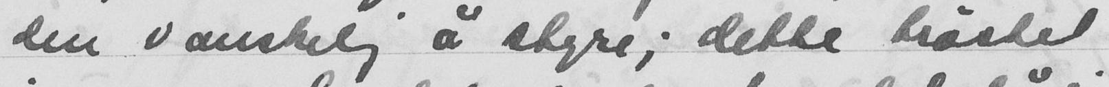den vanskelig å styre; dette trøstet
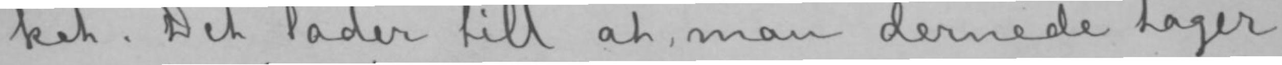ket. Det lader till at, man dernede tager
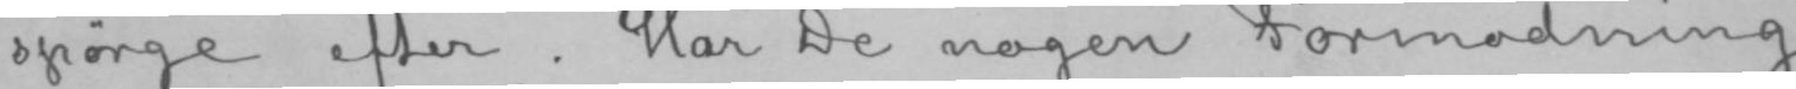spørge efter. Har De nogen Formodning
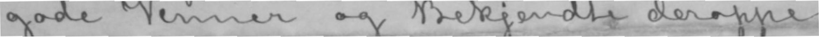gode Venner og Bekjendte deroppe
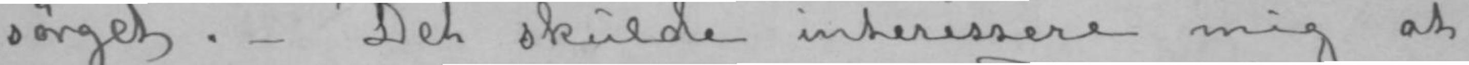sørget.- Det skulde interessere mig at
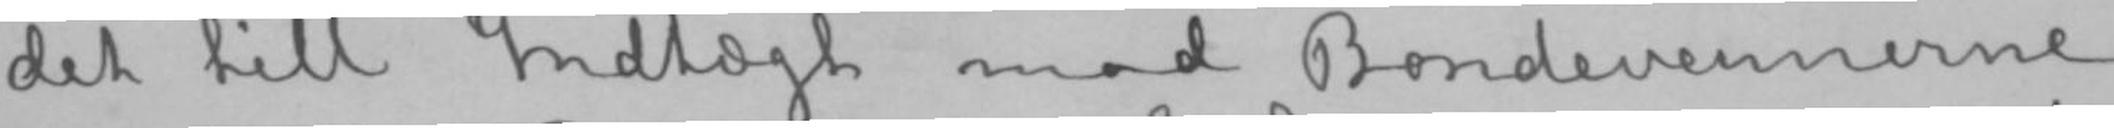det till Indtægt mod Bondevennerne
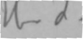H. I.
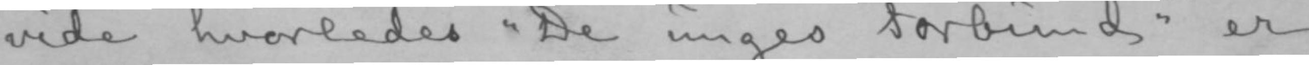vide hvorledes «De unges Forbund» er
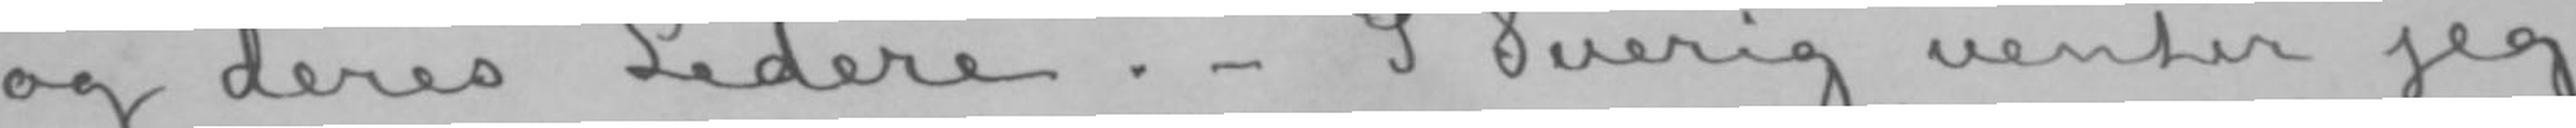og deres Ledere.- I Sverig venter jeg
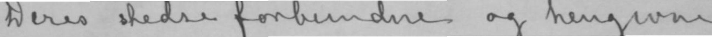Deres stedse forbundne og hengivne
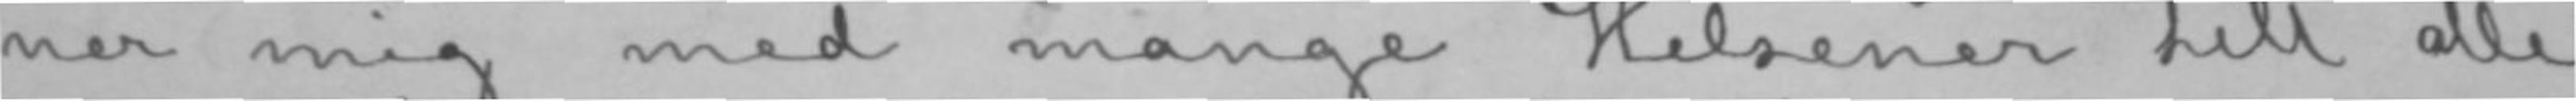ner mig med mange Hilsener till alle
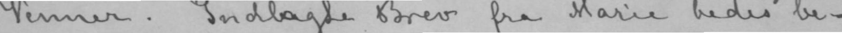Venner. Indlagte Brev fra Marie bedes be-
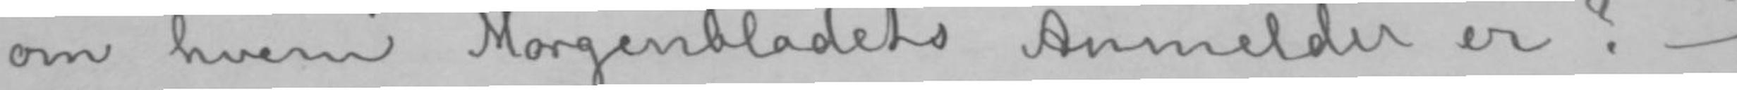om hvem Morgenbladets Anmelder er?-
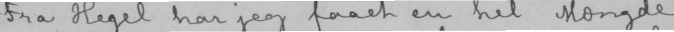Fra Hegel har jeg faaet en hel Mængde
